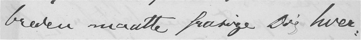breden maatte frasige Dig hver-
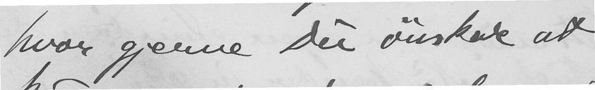hvor gjerne De ønskede at
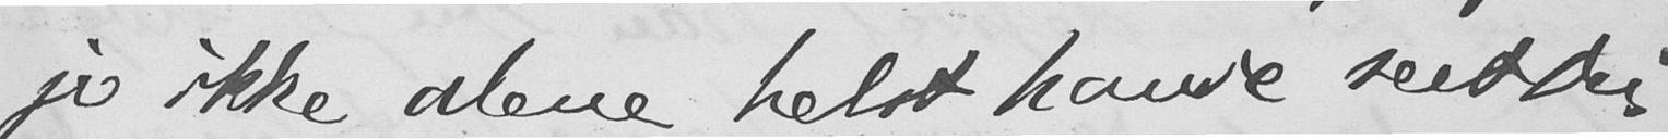jo ikke alene helst havde seet Dig
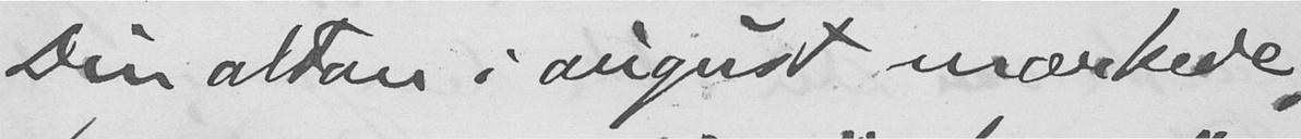Din altan i august mærkede
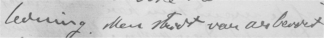ledning. Men stridt var arbeidet
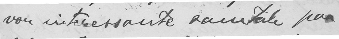vor interessante samtale paa
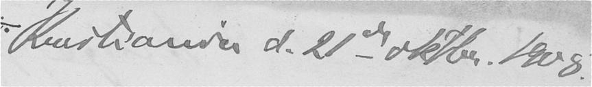Kristiania, d. 21de oktbr. 1908.
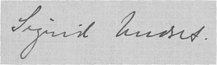Sigrid Undset.
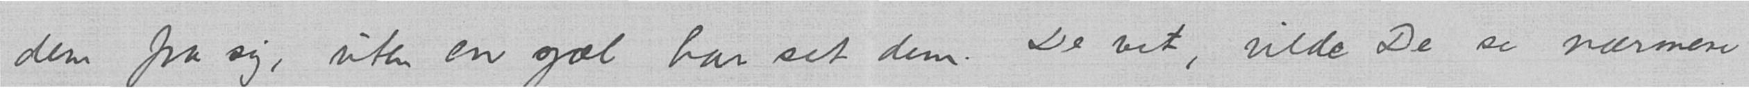dem fra sig, uten en sjæl har set dem. De vet, vilde De se nærmere
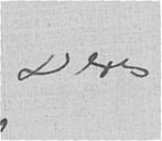Deres
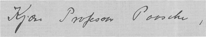Kjære Profssor Paasche,
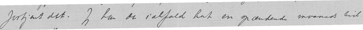fortjent det. Jeg har da ialfald hat en spændende maaneds tid
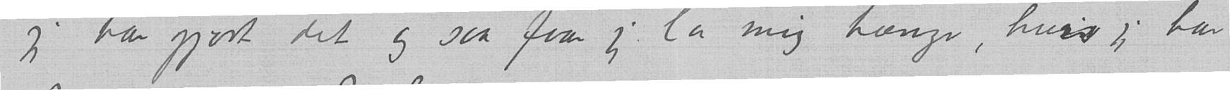jeg har gjort det og saa faar jeg la mig hænge, hvis jeg har
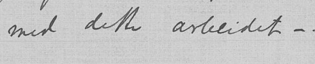med dette arbeidet -.
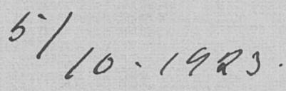5/10-1923.
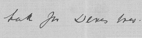tak for Deres brev.
